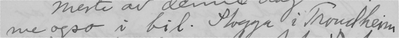me ogso i bil. Stogga i Trondheim
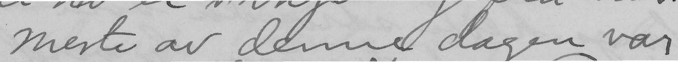meste av denne dagen var
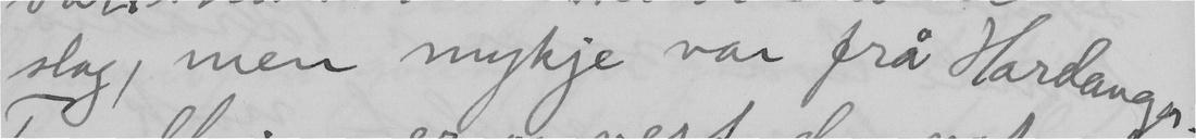slag, men mykje var frå Hardanger.
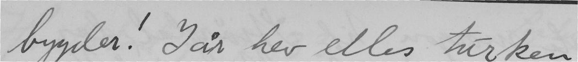bygder! I år hev elles turken
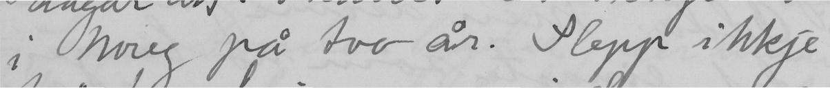i Noreg på tvo år. Slepp ikkje
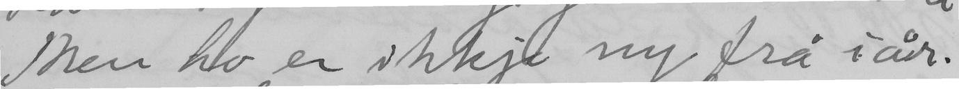Men ho er ikkje ny frå i år.
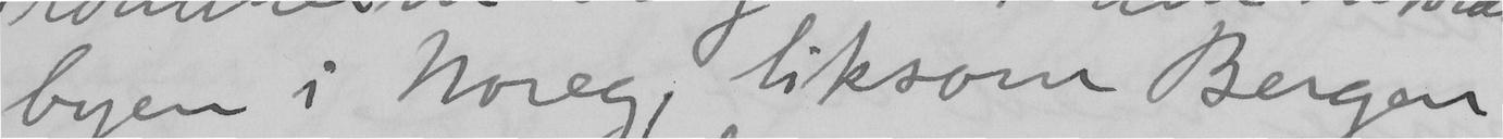byen i Noreg, liksom Bergen
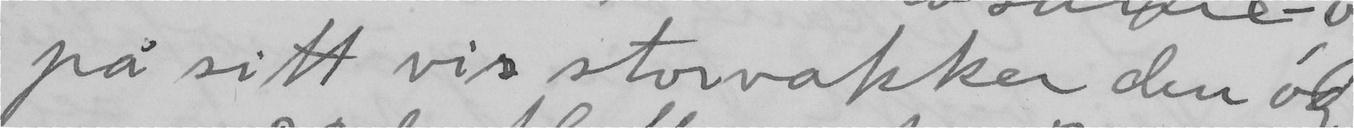på sitt vis storvakker den óg.
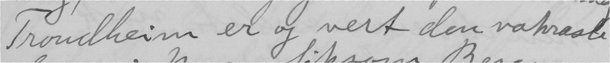Trondheim er og vert den vakraste
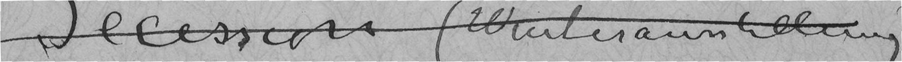Secession (Winterausstellung)
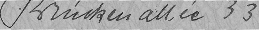Brücken allée 33
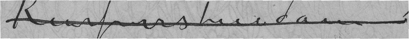Kurfurendam
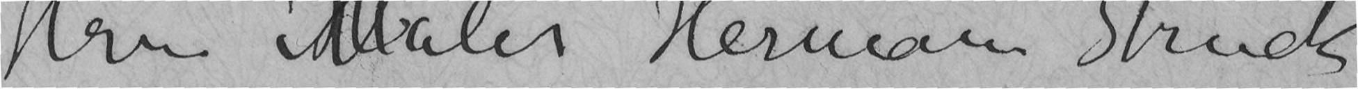Hrn HMaler Hermann Struck
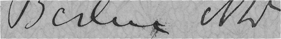Berlin NW
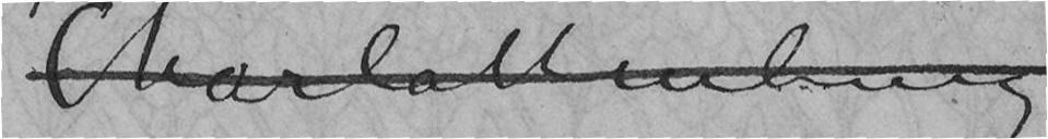Charlottenburg
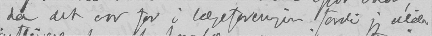da det var for i lægeforeningen fordi jeg vilde
